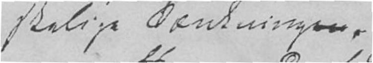skelige Tænkningen.
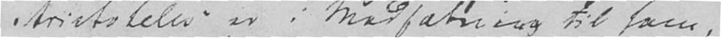Aristoteles er i Modsætning til ham,
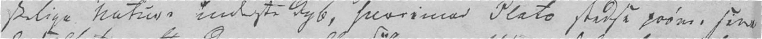skelige Naturs inderste Dyb, hvorimod Plato stedse prøver sine
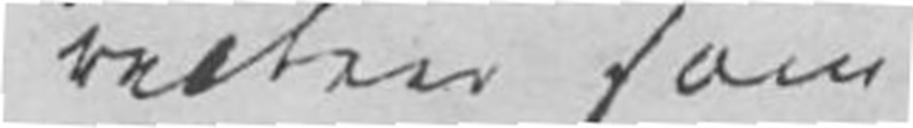racteer som
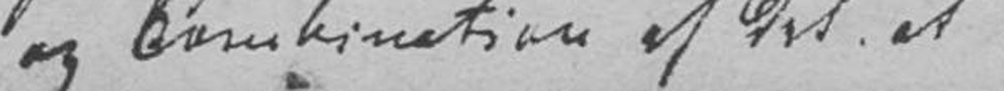og Combination af det at
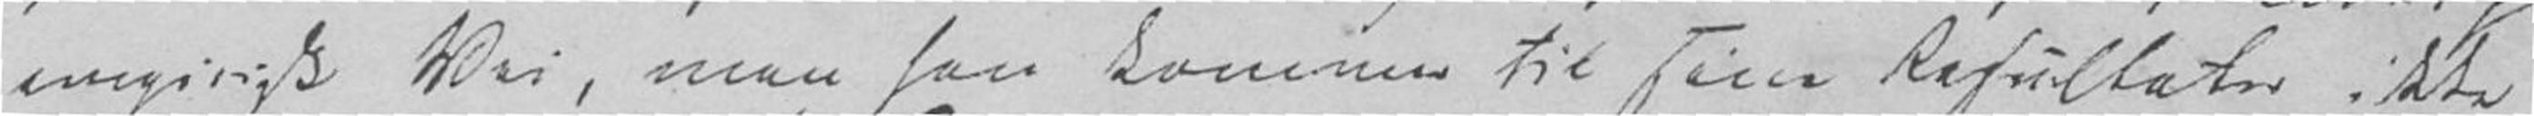empirisk Vei, men han kommer til sine Resultater ikke
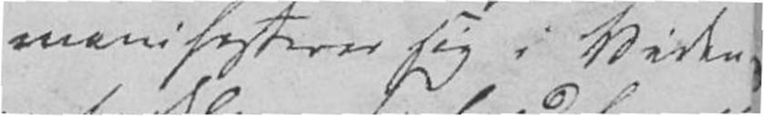manifesterer sig i Viden-
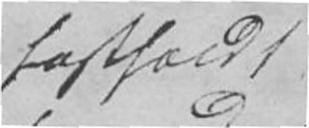fastholdt
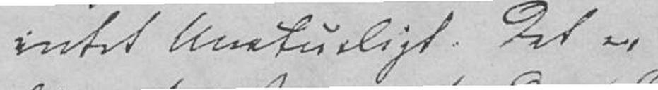intet Unaturligt. Det er
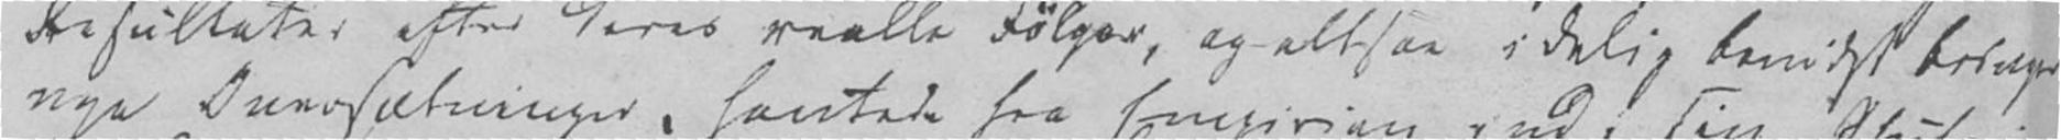Resultater efter deres reelle Følger, og altsaa idelig bevidst bringer
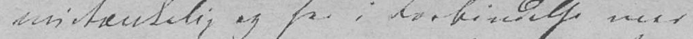mistænkelig og har i Forbindelse med
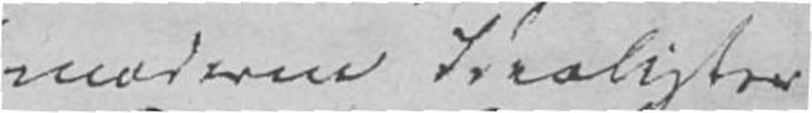moderne Idealister
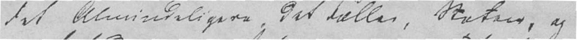det Almindeligere, det Fælles, Staten, og
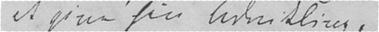at give sin Udvikling,
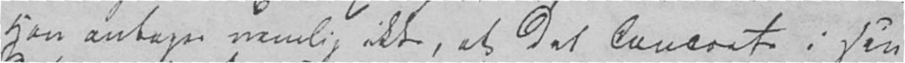Han antager nemlig ikke, at det Concrete i sin
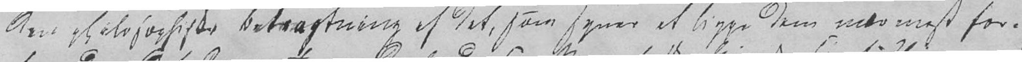den philosophiske Betragtning af det, som synes at ligge dem nærmest for-
